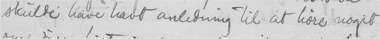skulde have havt anledning til at høre noget
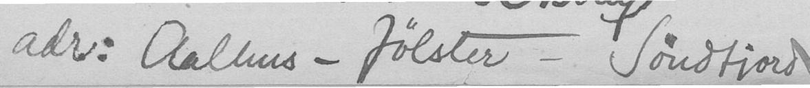adr: Aalhus - Jølster - Søndfjord
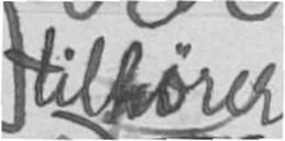tilhører
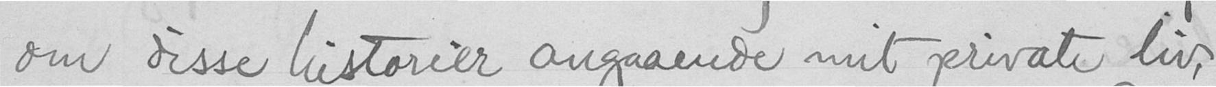om disse historier angaaende mit private liv,
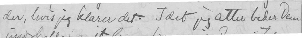der, hvis jeg klarer det. det jeg atter beder Dem
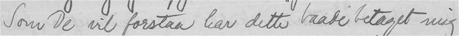Som De vil forstaa har dette baade betaget mig
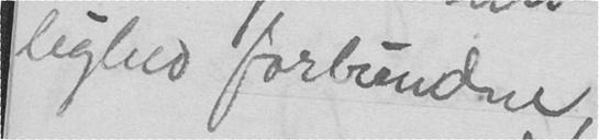lighed forbundne.
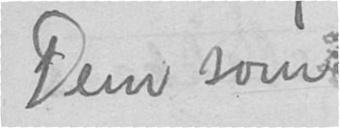Dem som
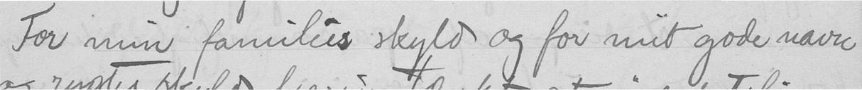For min families skyld og for mit gode navn
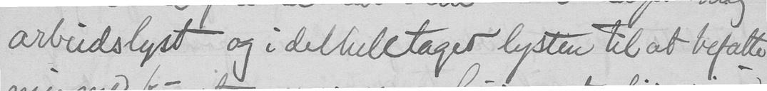arbeidslyst og i detheletaget lysten til at befatte
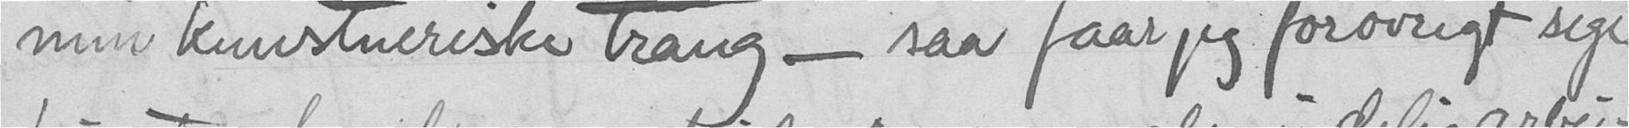min kunstneriske trang, - saa faar jeg forøvrigt sige
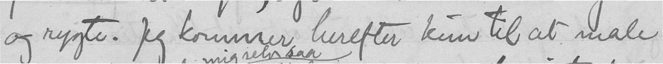og rygte. Jeg kommer herefter kun til at male
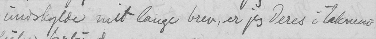undskylde mit lange brev, er jeg Deres i taknem-
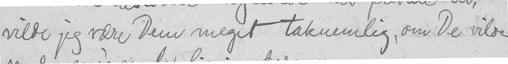vilde jeg være Dem meget taknemlig, om De vilde
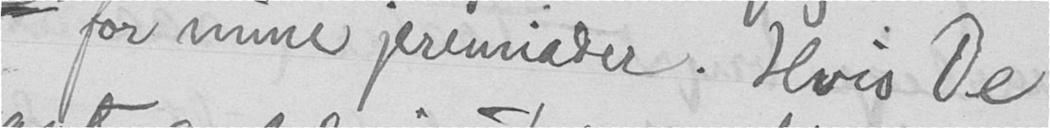for mine jeremiader. Hvis De
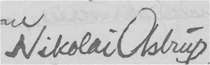Nikolai Astrup
